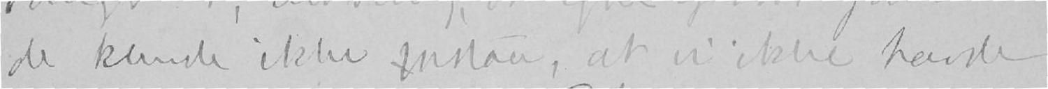de kunde ikke forstaa, at vi ikke havde
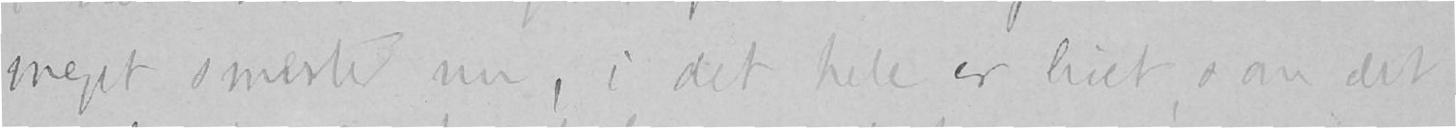meget smerte nu, i det hele er livet, som det
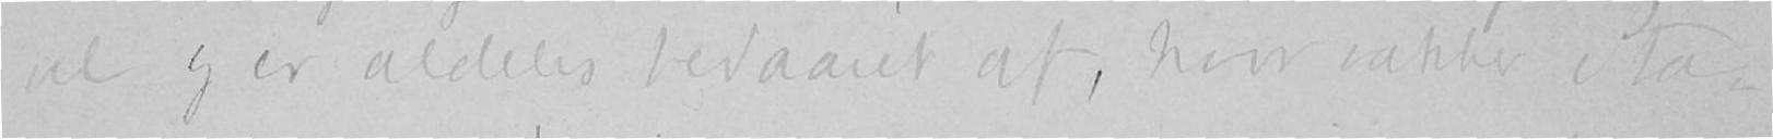vel og er aldeles bedaaret af, hvor vakker Sta-
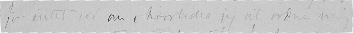jo intet ved om, hvorledes jeg vil ordne mig
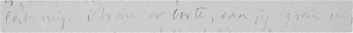lært mig. Strøm er borte, saa jeg greier mig
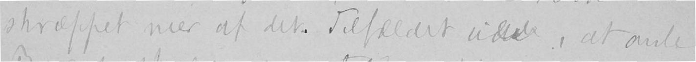skræppet mer af det. Tilfældet vilde, at oncle
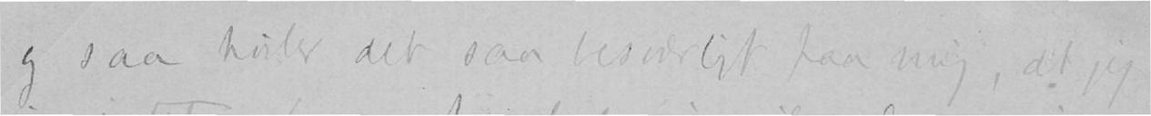og saa hviler det saa besværligt paa mig, at jeg
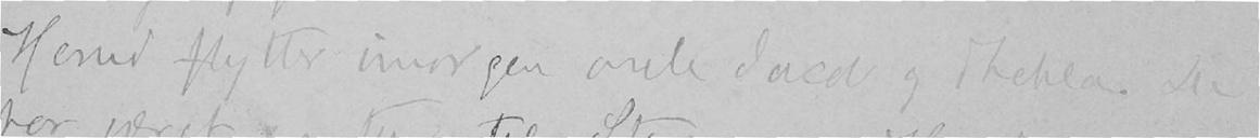Herud flytter imorgen oncle Jacob og Thekla. De
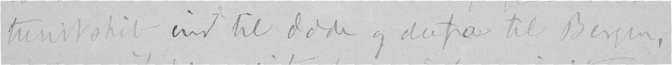turistskib ind til Odde og derfra til Bergen,
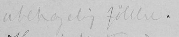ubehagelig følelse.
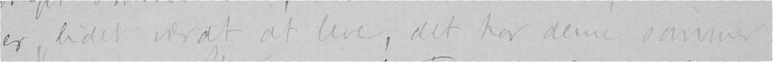er, lidet værdt at leve, det har denne sommer
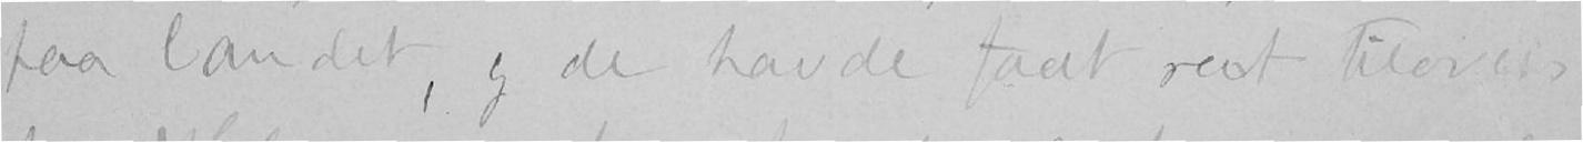paa landet, og de havde faat ræt tilovers
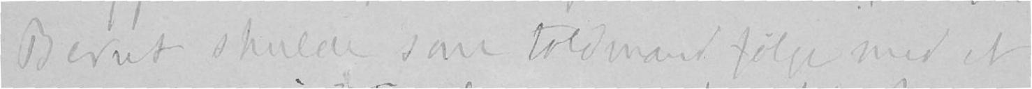Bernt skulde som toldmand følge med et
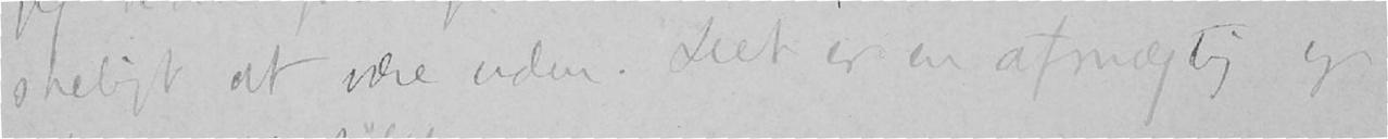skeligt at være uden. Det er en afmægtig og
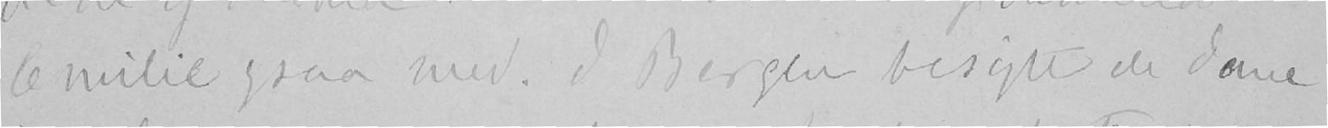Emilie ogsaa med. I Bergen besøgte de Jane
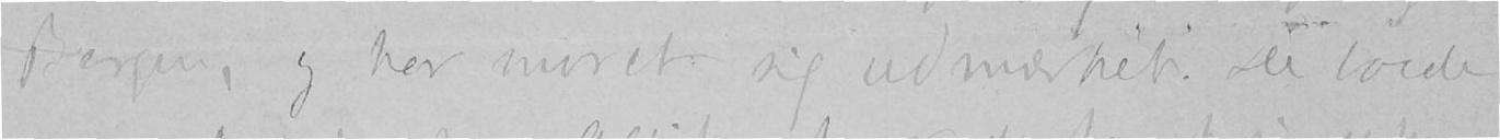Bergen, og har moret sig udmærket. De boede
